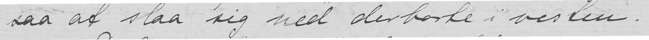saa at slaa sig ned der borte i vesten.
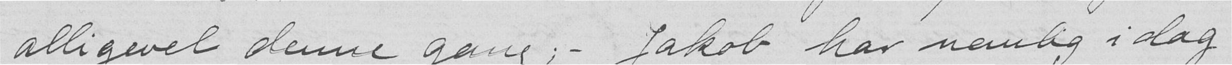alligevel denne gang; – Jakob har nemlig i dag
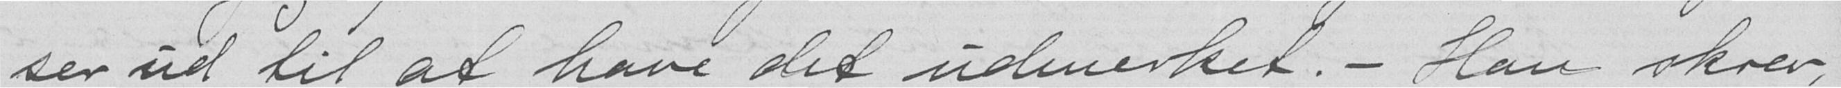ser ud til at have det udmerket. – Han skrev,
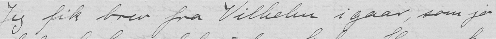Jeg fik brev fra Vilhelm igaar, som jo
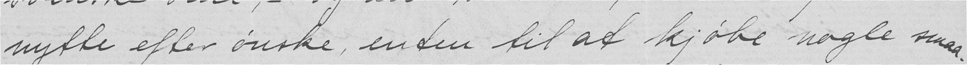nytte efter ønske, enten til at kjøbe nogle smaa-
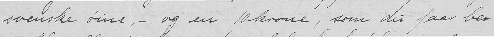svenske øine, – og en 10-krone, som du faar be-
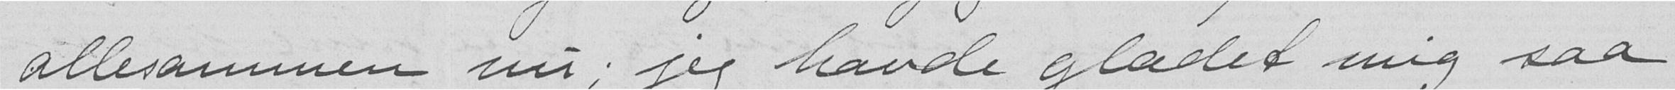allesammen nu; jeg havde glædet mig saa
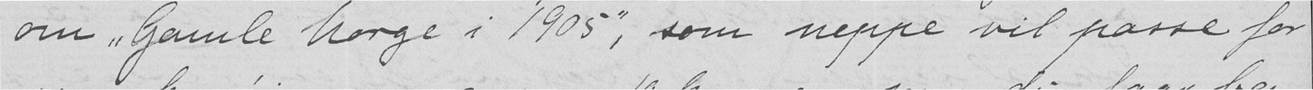om "Gamle Norge i 1905", som neppe vil passe for
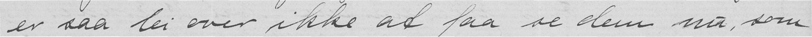er saa lei over ikke at faa se dem nu, som
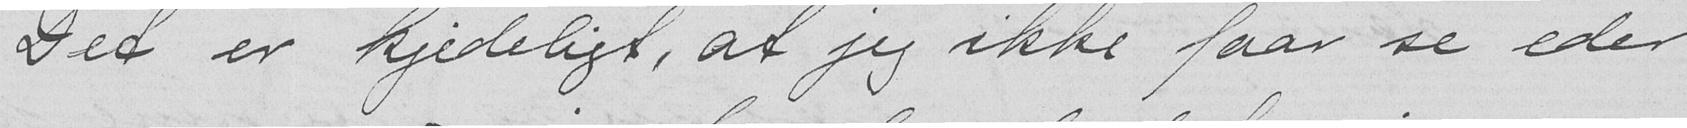Det er kjedeligt, at jeg ikke faar se eder
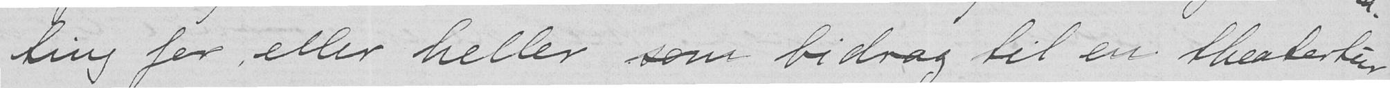ting for, eller heller som bidrag til en theatertur
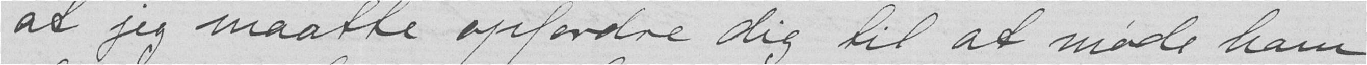at jeg maatte opfordre dig til at møde ham
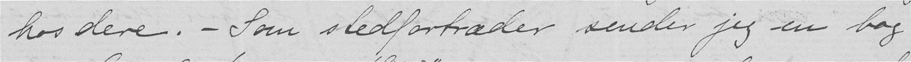hos dere. – Som stedfortræder sender jeg en bog
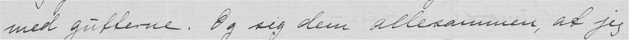med gutterne. Og sig dem allesammen, at jeg
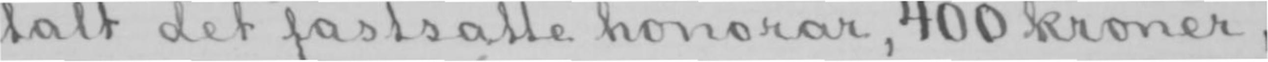talt det fastsatte honorar, 400 kroner,
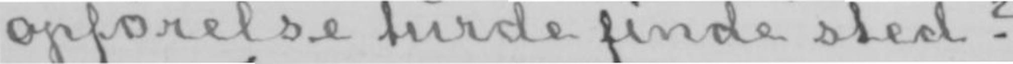opførelse turde finde sted?
